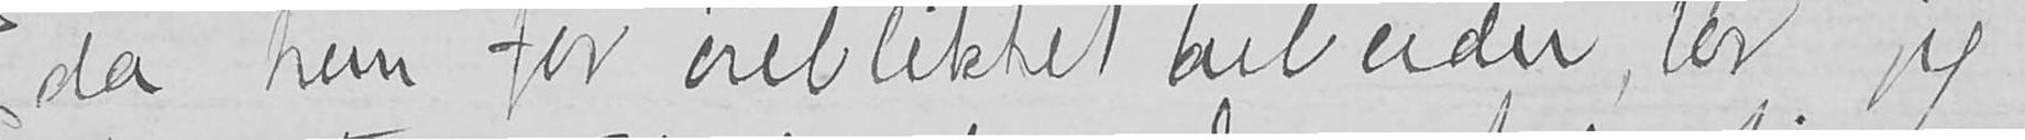da hun for øieblikket arbeider, tør jeg
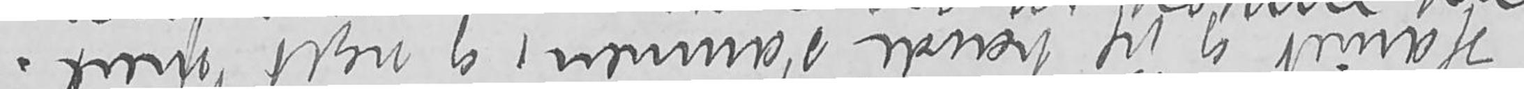Harriet og jeg havde sammen, og noget mere.
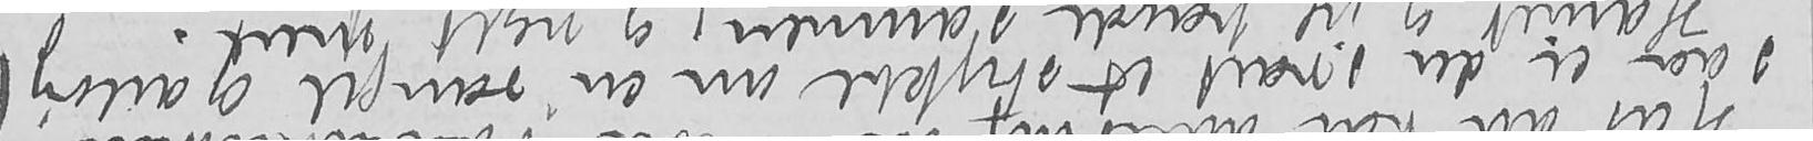saa er der snart et stykke om en rangel Garborg
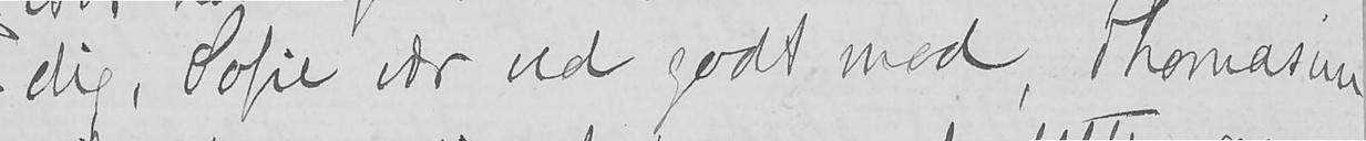dig, Sofie var ved godt mod, Thomasine
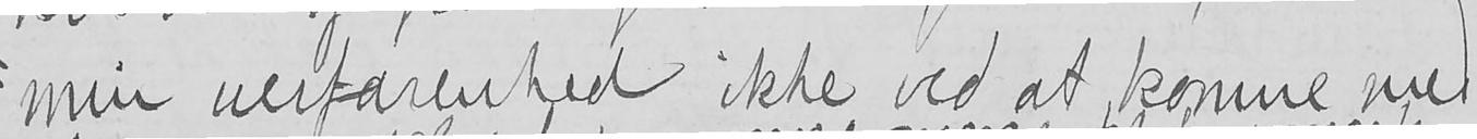min uerfarenhed ikke ved at komme med.
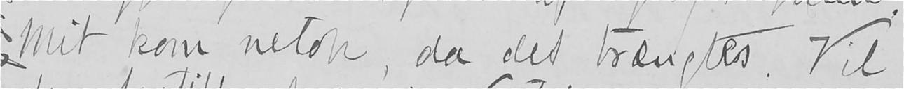Mit kom netop, da det trængtes. Vil
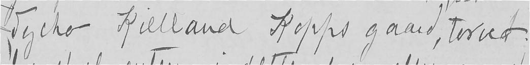Tycho Kielland Kopps gaard, torvet.
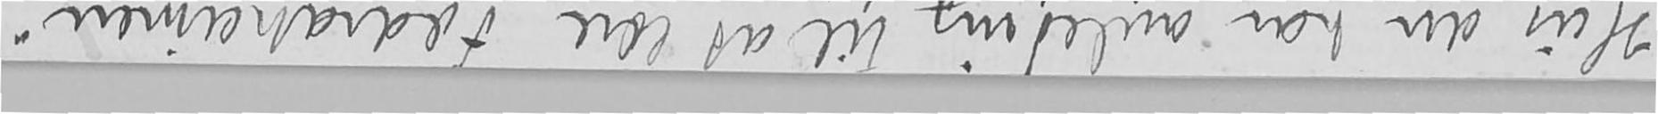Hvis du har anledning til at læse "Fædraheimen",
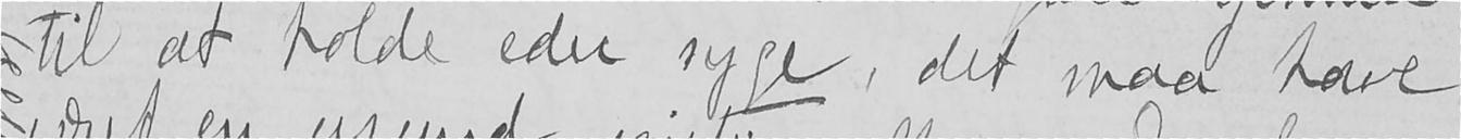til at holde eder syge, det maa have
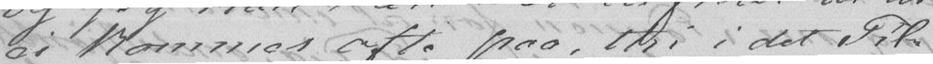ei kommer ofte paa, thi i det Til-
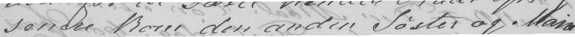senere kom den anden Søster og Marie
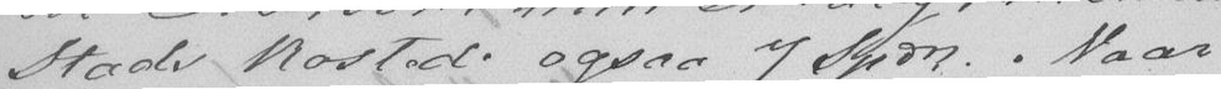Stads kostede ogsaa 7 Spd.. Naar
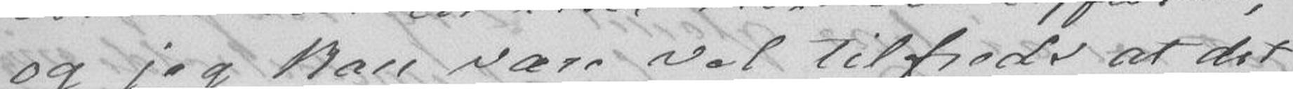og jeg kan være vel tilfreds at det
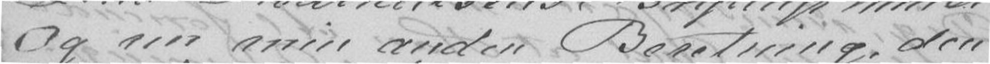Og nu min anden Beretning den
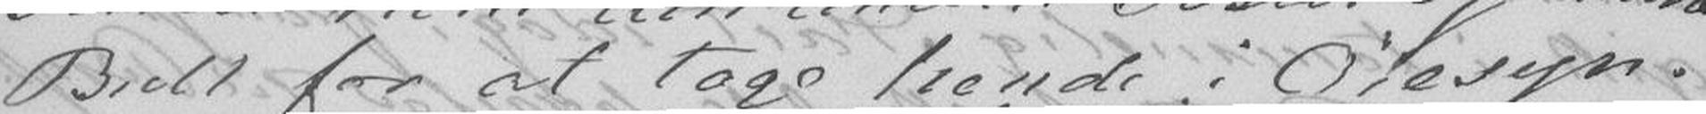Bull for at tage hende i Øiesyn.
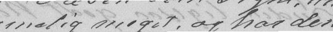melig meget, og har der-
